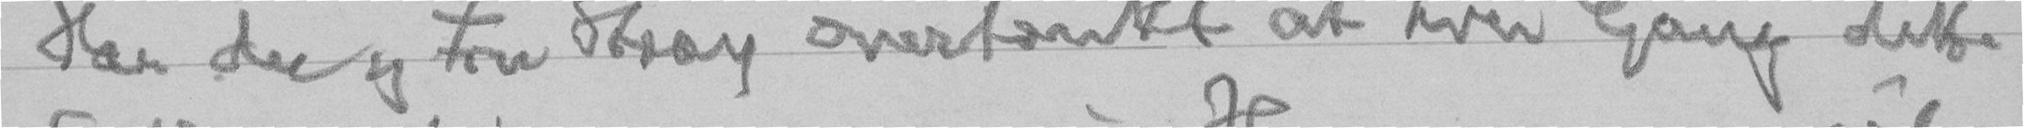Har du og Fru Stray overtænkt at hver Gang dette
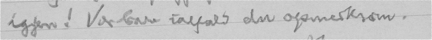igjen! Vær bare ialfald du opmerksom.
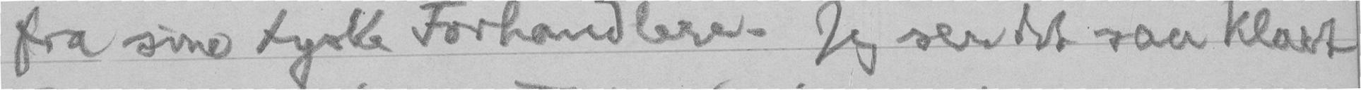fra sine tyske Forhandlere. Jeg ser det saa klart
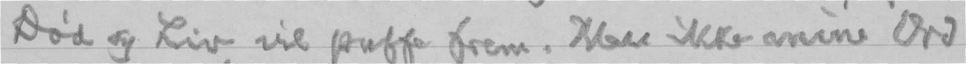Død og Liv vil puffe frem. Men ikke mine Ord
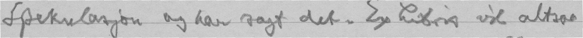Spekulasjon og har sagt det. Ex Libris vil altsaa
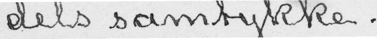dels samtykke.
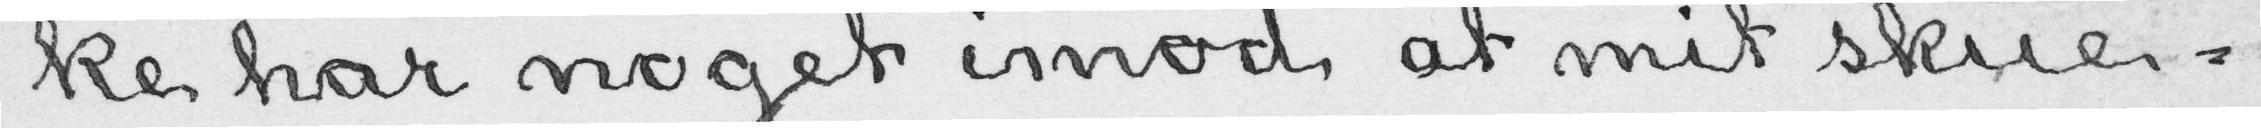ke har noget imod at mit skue-
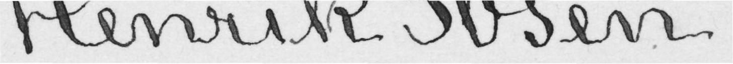Henrik Ibsen.
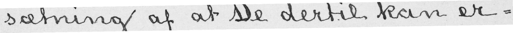sætning af at De dertil kan er-
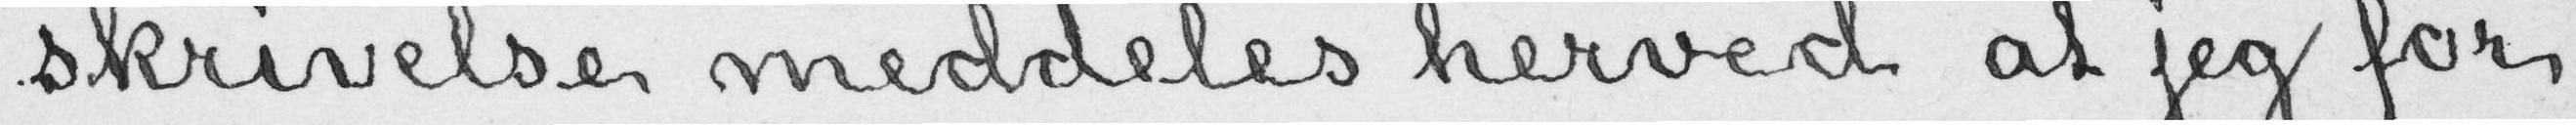skrivelse meddeles herved at jeg for
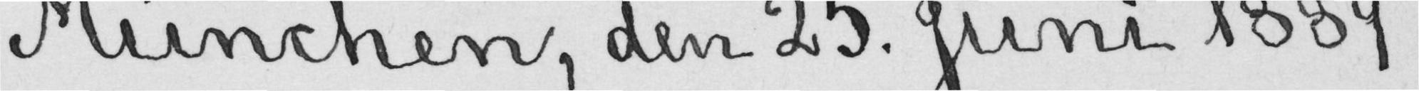München, den 25. Juni 1889.
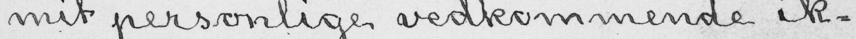mit personlige vedkommende ik-
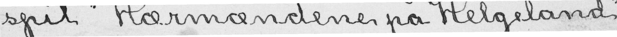spil «Hærmændene på Helgeland»
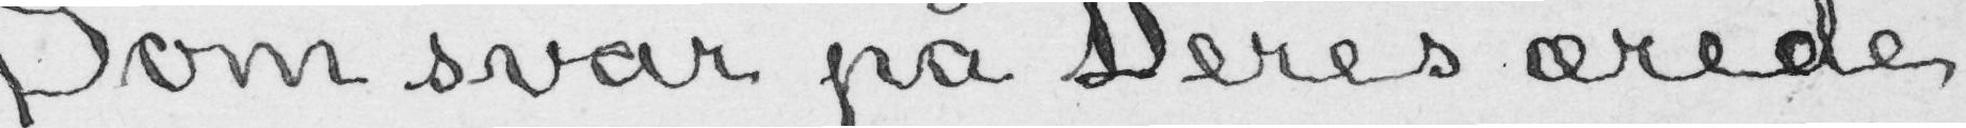Som svar på Deres ærede
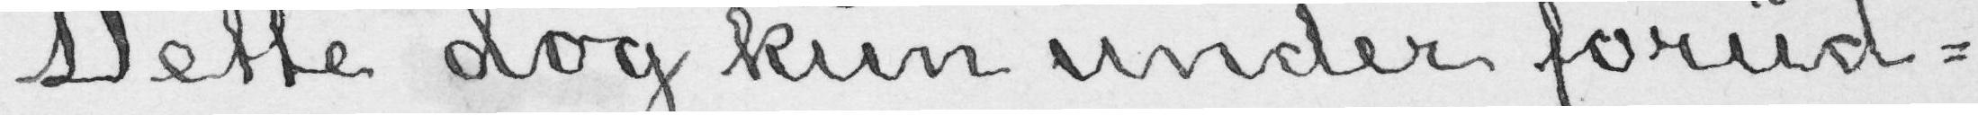Dette dog kun under forud-
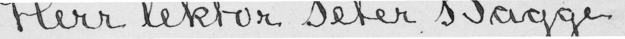Herr lektor Peter Bagge.
